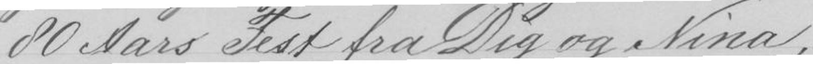80 Aars Fest fra Dig og Nina,
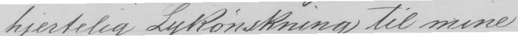hjertelig Lykønskning til mine
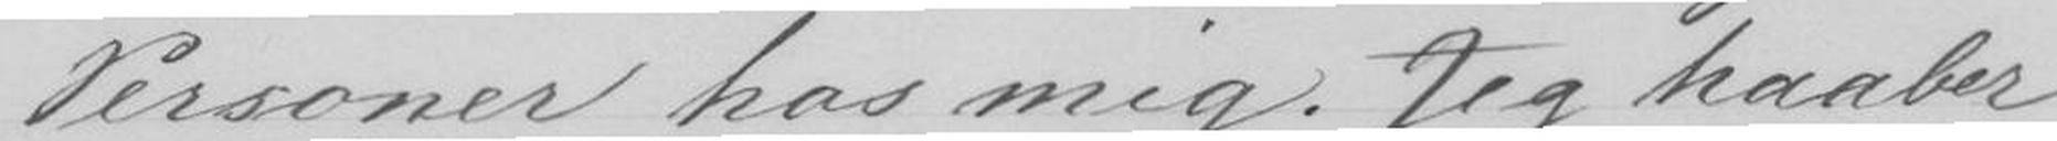Personer hos mig, Jeg haaber
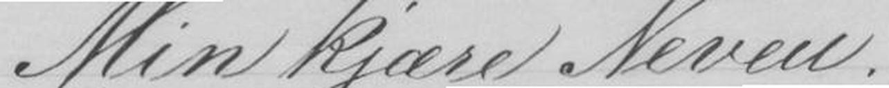Min kjære Neveu!
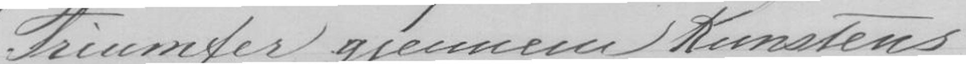Triumfer gjennem Kunstens
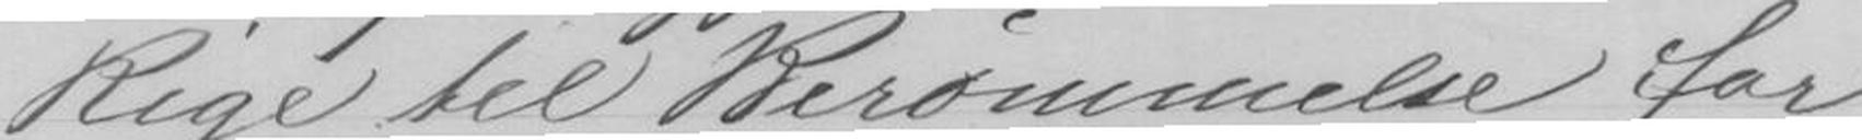Rige til Berømmelse for
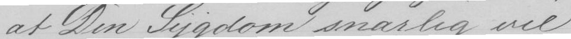at Din Sygdom snarlig vil
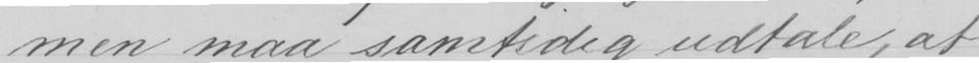men maa samtidig udtale, at
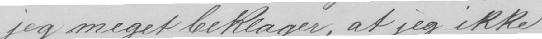jeg meget beklager, at jeg ikke
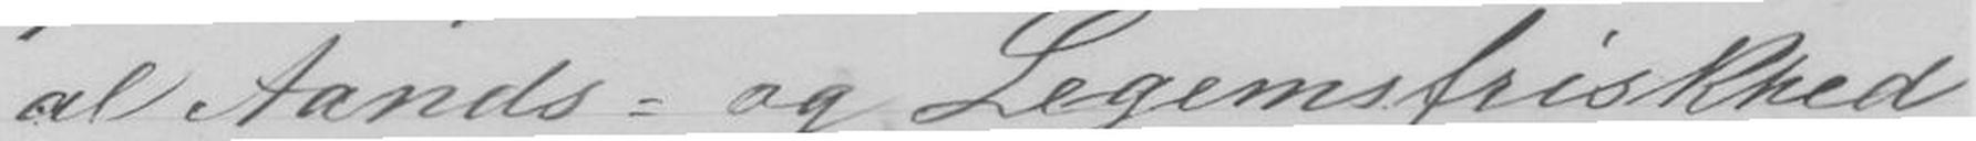al Aands = og Legemsfriskhed
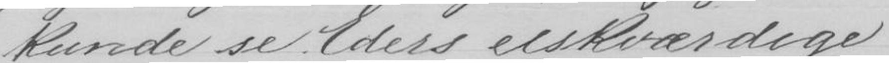kunde se Eders elskværdige
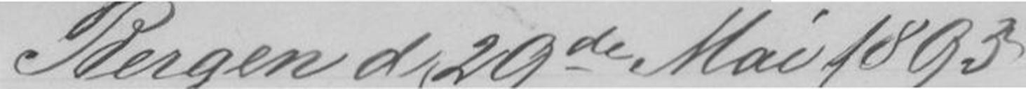Bergen d 29de Mai 1893.
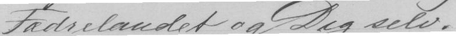Fædrelandet og Dig selv
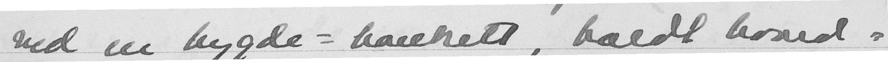ved en bygde-bankett, holdt hoved-
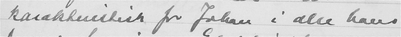karakteristisk for Johan i alle hans
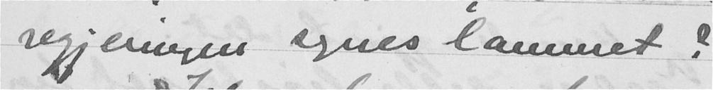regjeringen synes lammet?
